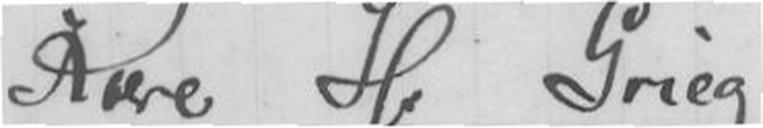Kære Hr Grieg.
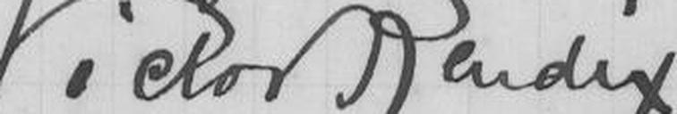Victor Bendix
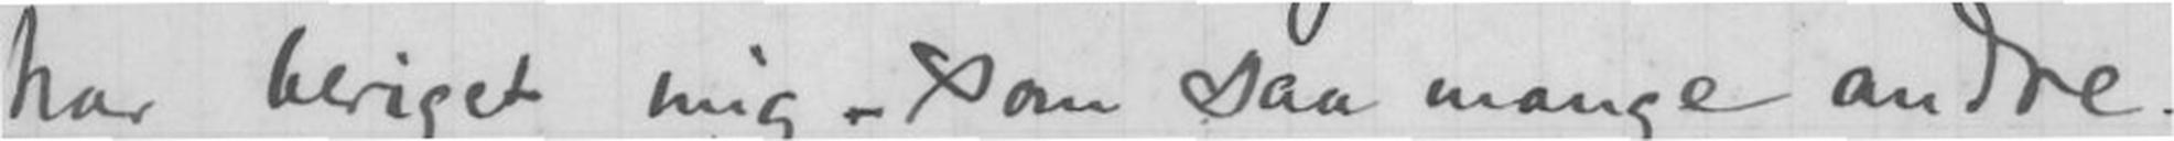har beriget mig - som saa mange andre.
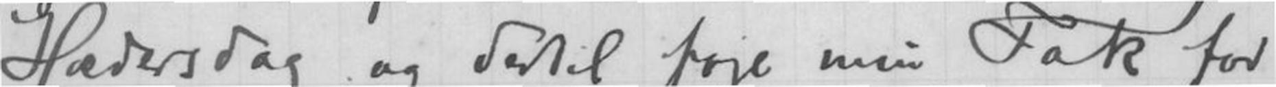Hædersdag og dertil føje min Tak for
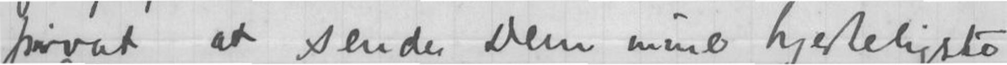privat at sende Dem mine hjerteligste
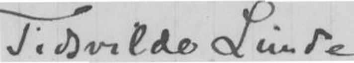Tidsvilde Lunde
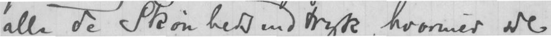alle de Skønhedsindtryk, hvormed De
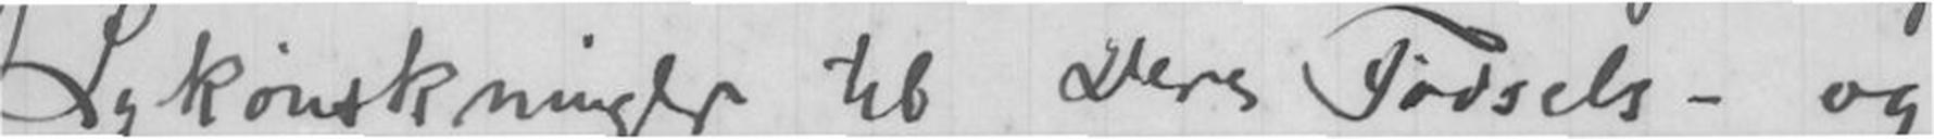Lykønskninger til Deres Fødsels- og
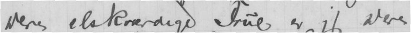Deres elskværdige Frue er jeg Deres,
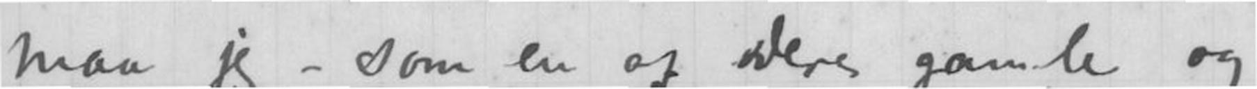maa jeg - som en af Deres gamle og
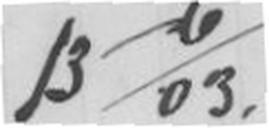13-6/03.
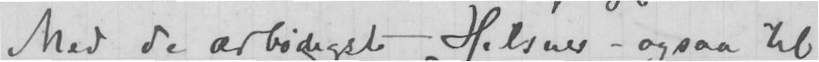Med de ærbødigste - Hilsner - ogsaa til
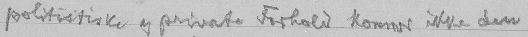politistiske og private Forhold kommer ikke den
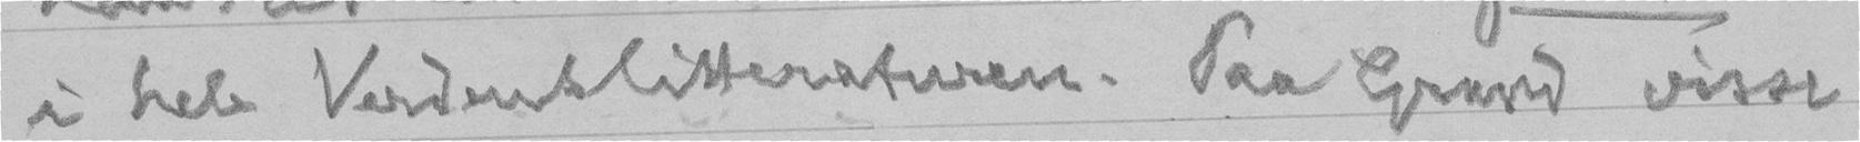i hele Verdenslitteraturen. Paa Grund visse
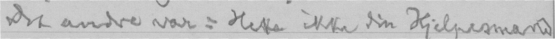Det andre var: Hette ikke din Hjelpesmand
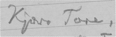Kjære Tore,
